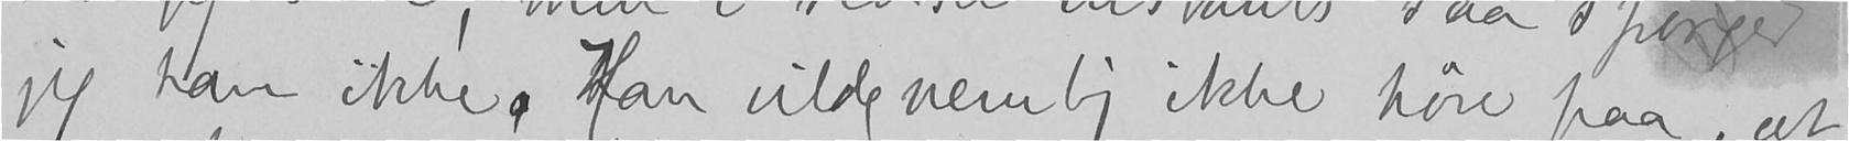jeg ham ikke. Han vilde nemlig ikke høre paa, at
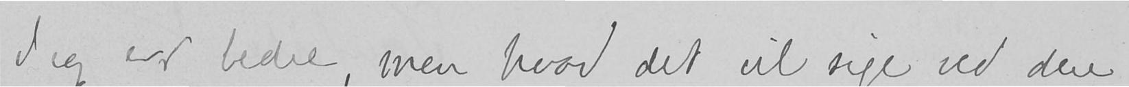Jeg er bedre, men hvad det vil sige, ved dere
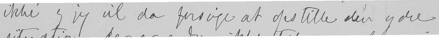ikke og jeg vil da forsøge at opstille den ydre
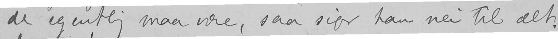de egentlig maa være, saa siger han nei til det.
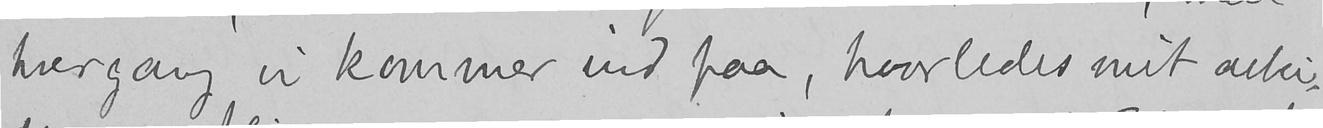hvergang vi kommer ind paa, hvorledes mit arbei-
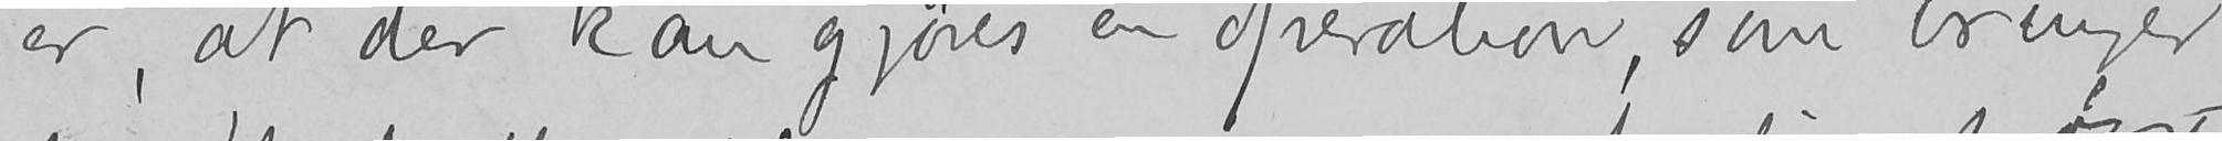er, at der kan gjøres en operation, som bringer
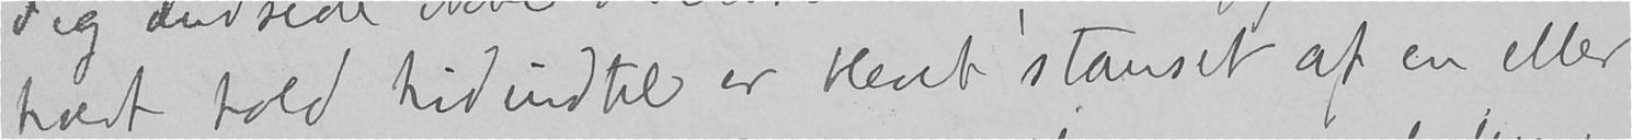hvert hold hid indtil er blevet stanset af en eller
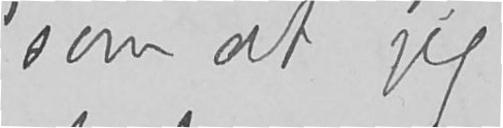som at jeg
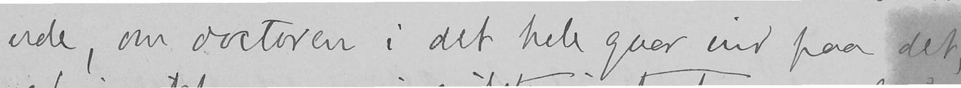ude, om doctoren i det hele gaar ind paa det,
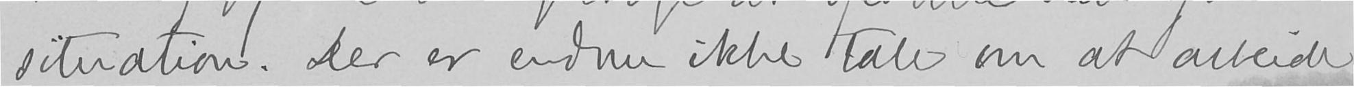situation. Der er endnu ikke tale om at arbeide
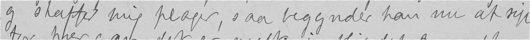og skaffer mig plager, saa begynder han nu at sige
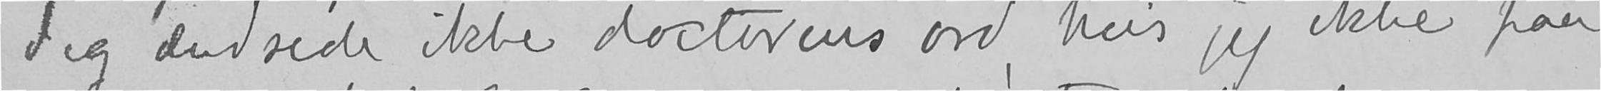Jeg ændrede ikke doctorens ord, hvis jeg ikke paa
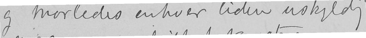og hvorledes enhver liden uskyldig
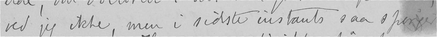ved jeg ikke, men i sidste instants saa spørger
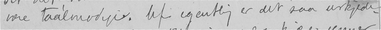være taalmodige. Uf egentlig er det saa urkjede-
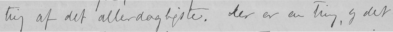ting af det allerdagligste. Der er en ting, og det
